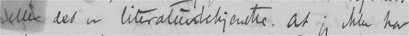eller det er literaturbekjendte. At jeg ikke har
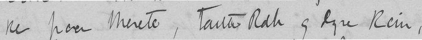ke paa Merete, tante Rah og dyre Rein,
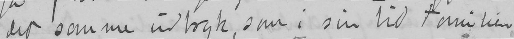det samme indtryk, som i sin tid Familien
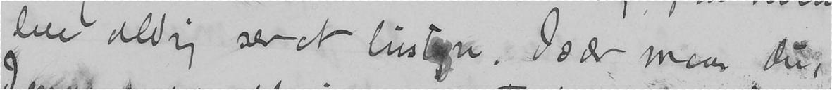dere aldrig ser et livstegn. Især maa du,
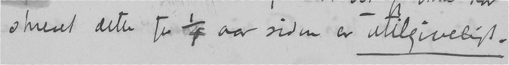skrevet dette for ¼ aar siden er utilgiveligt.
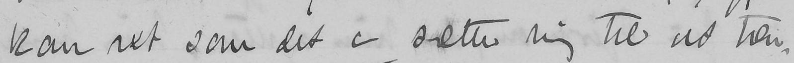kan ret som det er sætte mig til at tæn-
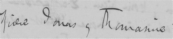Kjære Jonas og Thomasine
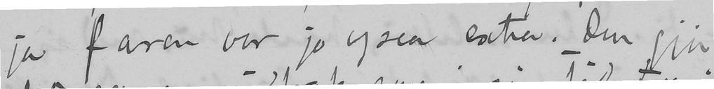ja faren var jo ogsaa extra. Den gjør
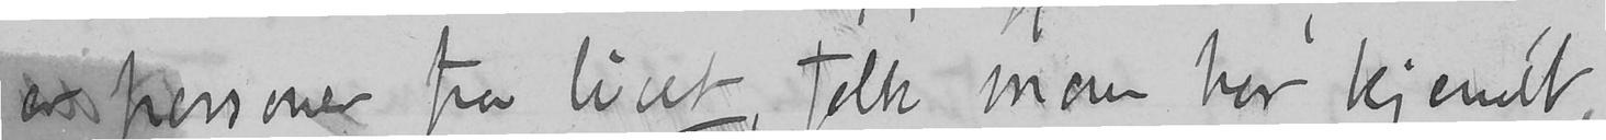er personer fra livet, folk man har kjendt,
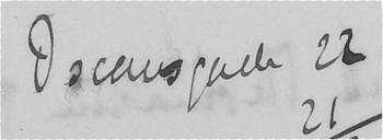Oscarsgade 22
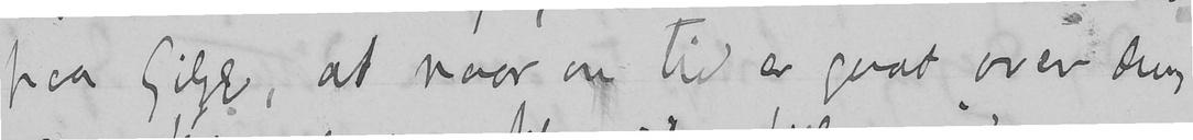paa Gilje, at naar en tid er gaat over dem
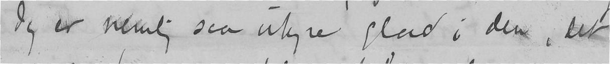Jeg er nemlig saa uhyre glad i den, det
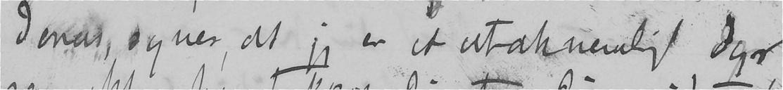Jonas, synes, at jeg er et utaknemligt dyr
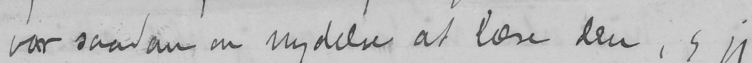var saadan en nydelse at læse den, og jeg
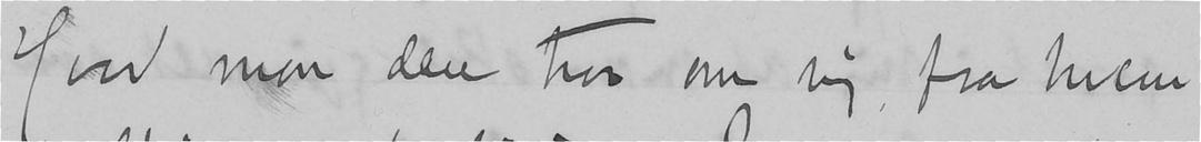Hvad mon dere tror om mig, fra hvem
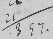21/3 97.
